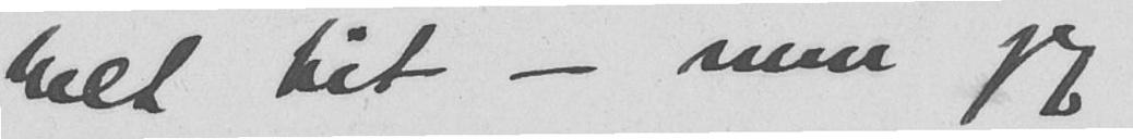helt hit - men jeg
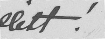slett
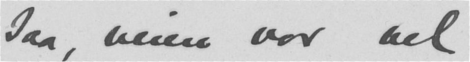saa, veien var hel
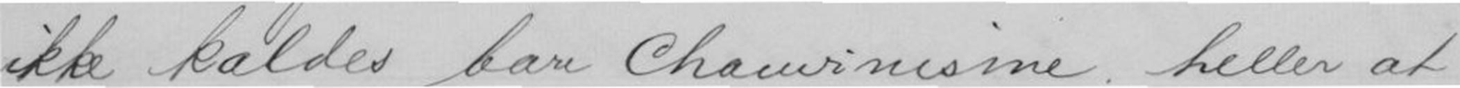ikke kaldes bare chauvinisme heller at
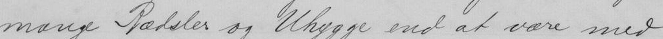mange Rædsler og Uhygge end at være med
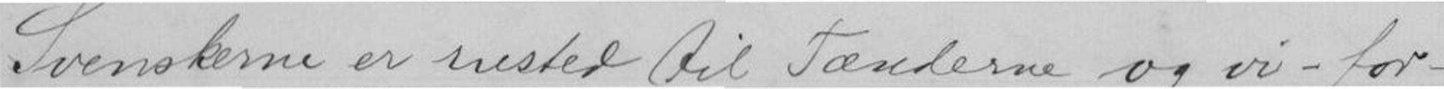Svenskerne er rustet til Tænderne og vi - for-
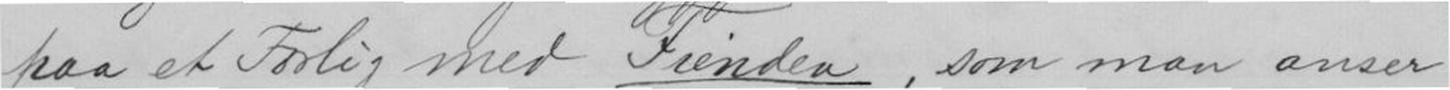paa et Forlig med Fienden, som man anser
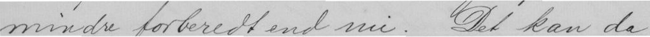mindre forberedt end nu. Det kan da
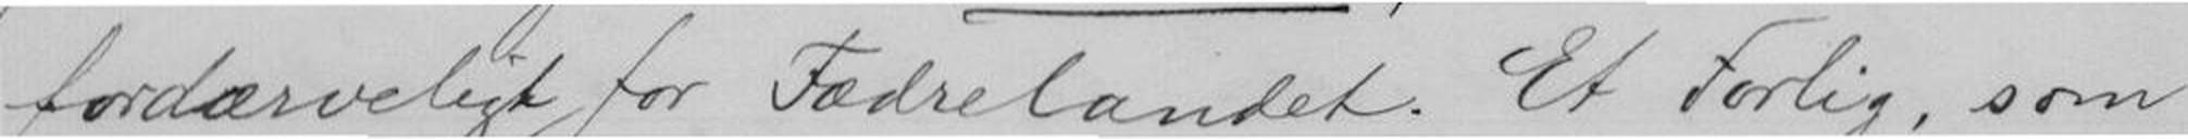fordærveligt for Fædrelandet. Et forlig, som
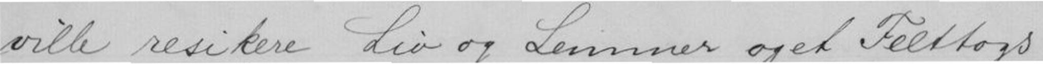ville resikere Liv og Lemmer og et Felttogs
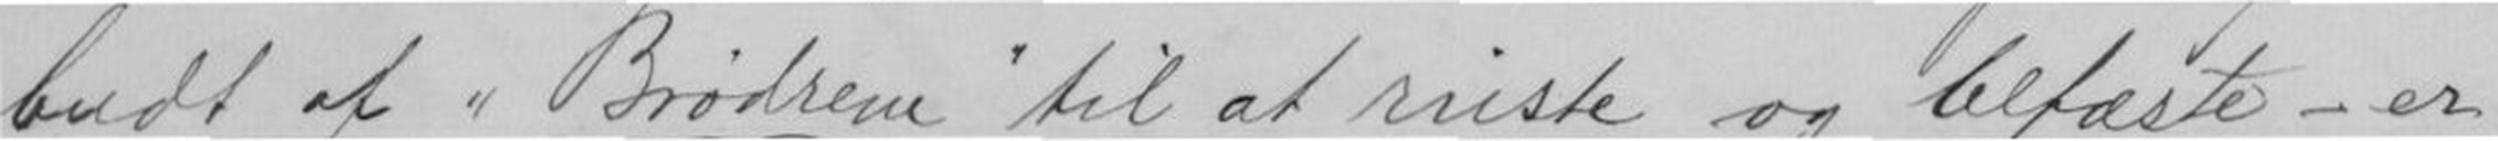budt af Brødrene til at ruste og befæste - er
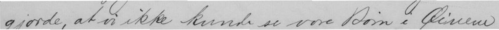gjorde, at vi ikke kunde se vore Børn i Øinene,
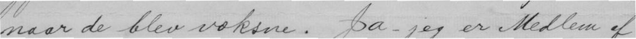naar de blev voksne. Ja - jeg er Medlem af
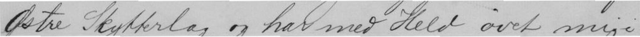Østre Skytterlag og har med Held øvet mig i
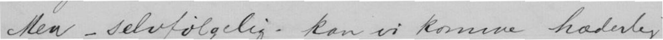Men - selvfølgelig, kan vi komme hæderlig
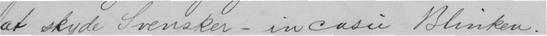at skyde Svensker - in casu Blinken.
